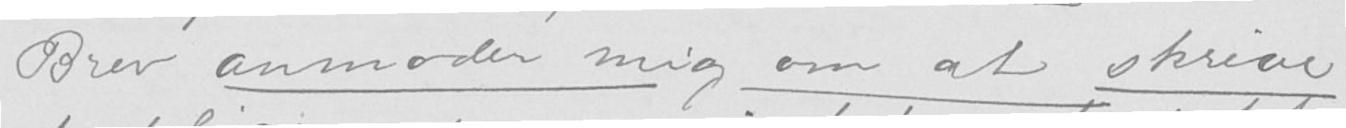Brev anmoder mig om at skrive
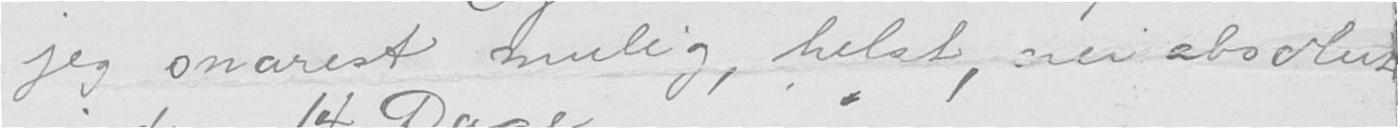jeg snarest mulig, helst, nei absolut
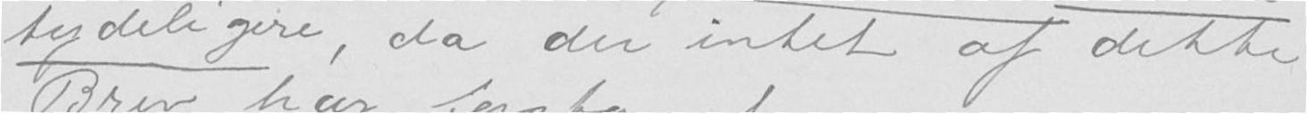tydeligere, da du intet af dette
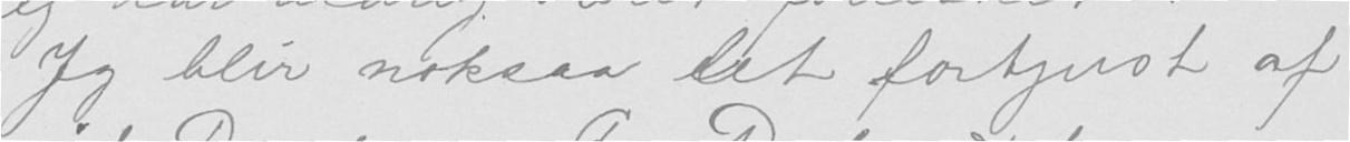Jeg blir noksaa let fortjust af
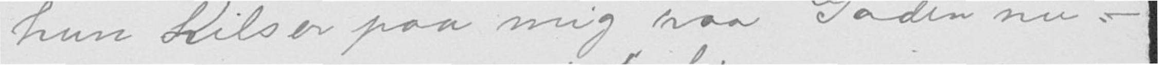hun hilser paa mig paa Gaden nu -
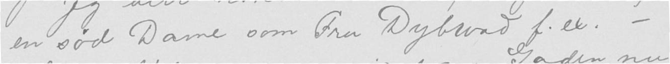en sød Dame som Fru Dybwad f.ex. -
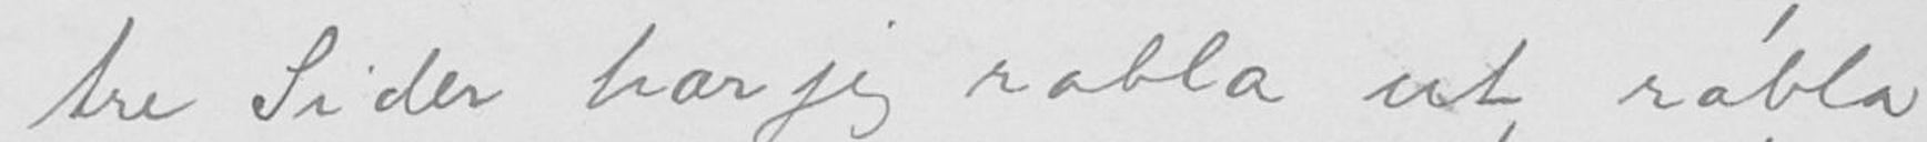tre Sider har jeg rabla ut, rabla
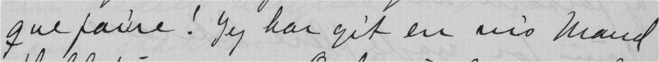que faire! Jeg har git en vis Mand
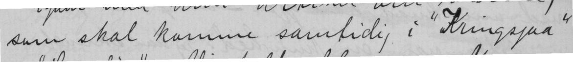som skal komme samtidig i "Kringsjaa"
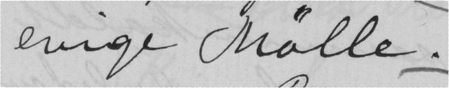evige mølle.
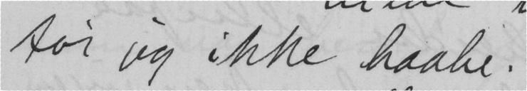tør jeg ikke haabe.
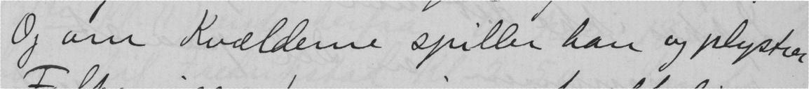Og om Kvælderne spiller han og plystrer
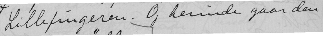Lillefingeren. Og herinde gaar den
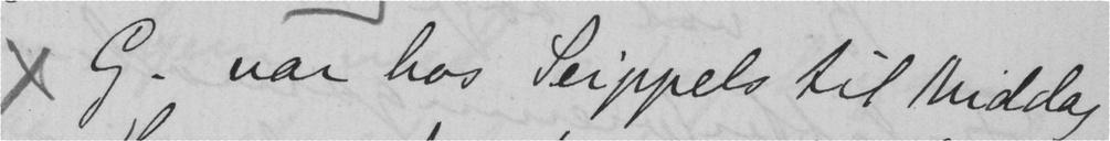x G. var hos Seippels til middag
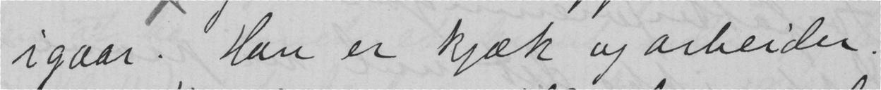igaar. Han er kjæk og arbeider.
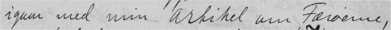igaar med min Artikel om Færøerne,
